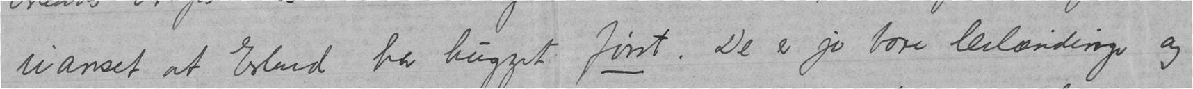uanset at Erlend har hugget først. De er jo bare leilændinge og
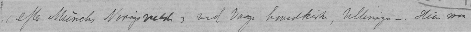(efter Munchs Norigsvælde) ved Vaage hovedkirke, Ullinsyn –. Hun maa
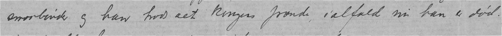smaabønder og han trods alt kongens frænde, ialfald nu han er død.
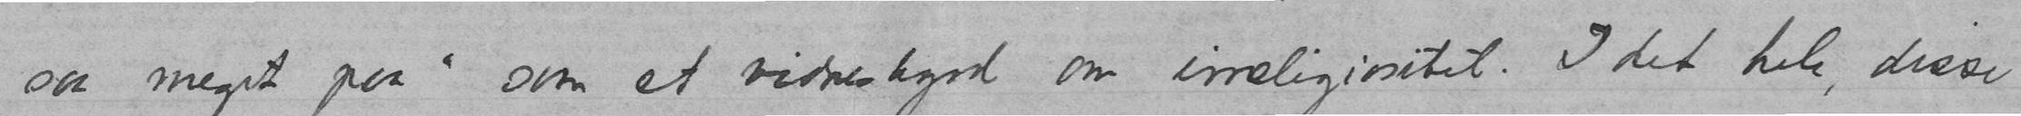saa meget paa" som et vidnesbyrd om irreligiøsitet. I det hele, disse
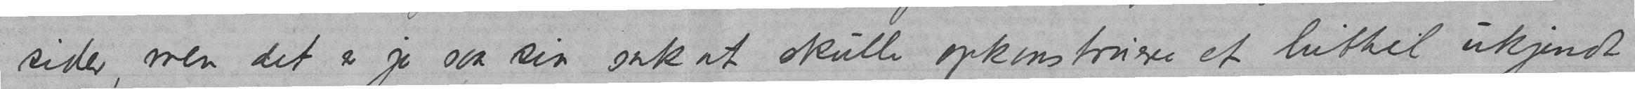sider, men det er jo saa sin sak at skulle opkonstruere et hittil ukjendt
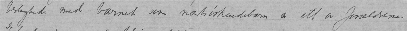beslegtede med barnet som næstsøskendebarn av ett av forældrene.
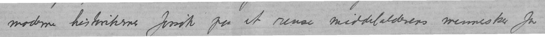moderne historikernes forsøk paa at rense middelalderens mennesker for
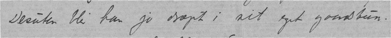Desuten blir han jo dræpt i sit eget gaardstun.
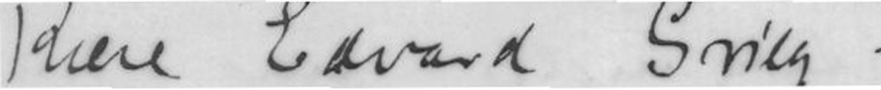Kære Edvard Grieg!
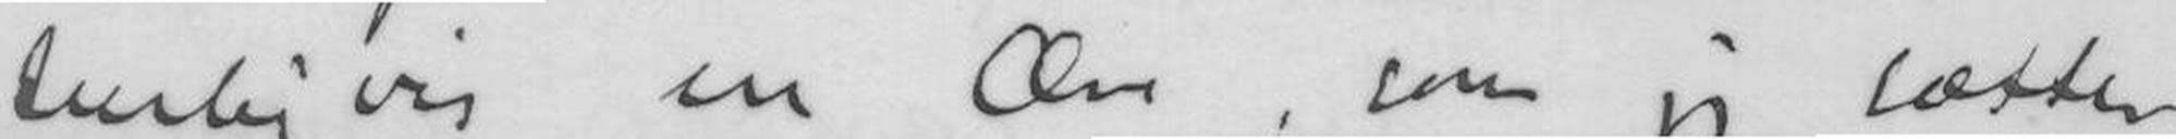turligvis en Ære som jeg sætter
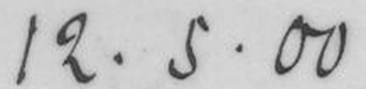12.5.00
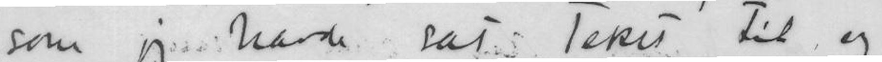som jeg havde sat Tekst til og
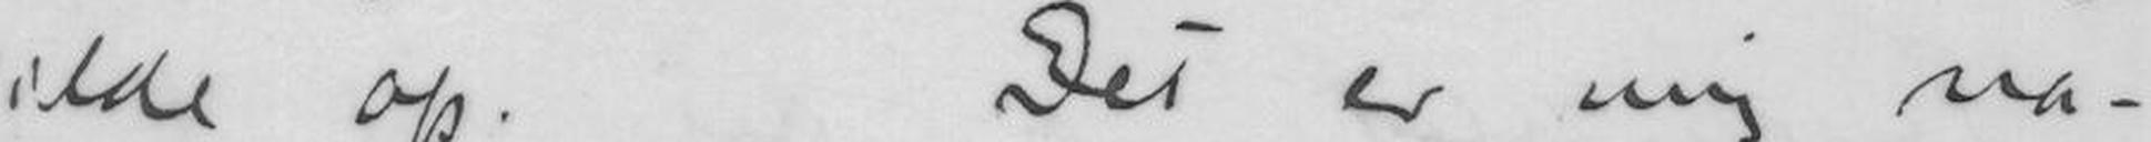ilde op. Det er mig na-
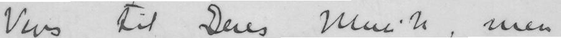Vers til Deres Musik, men
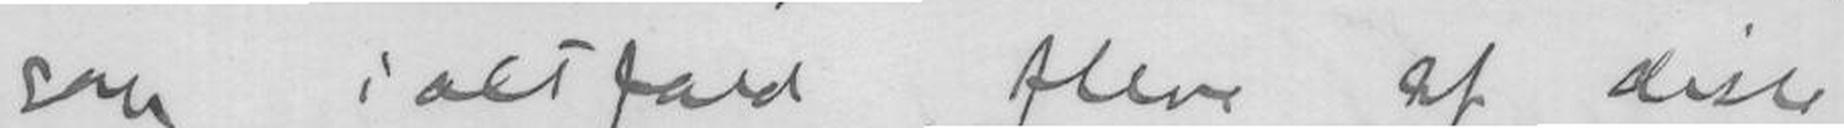som ialtfald flere af disse
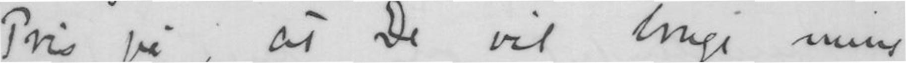Pris på, at De vil bruge mine
